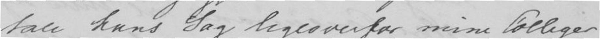tale hans Sag ligeoverfor mine Colleger
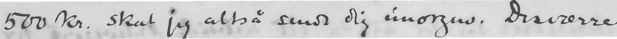500 Kr, skal jeg altså sende dig imorgen. Desværre
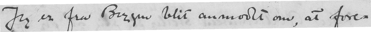Jeg er fra Bergen blit anmodet om, at fore-
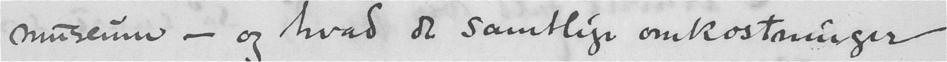museum - og hvad de samtlige omkostninger
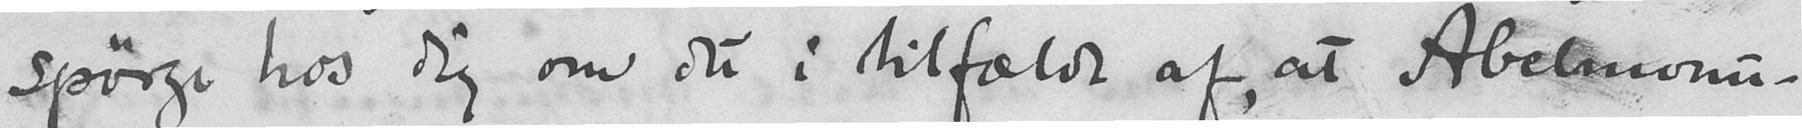spørge hos dig om det i tilfælde af, at Abelmonu-
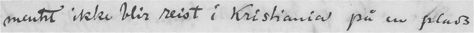mentet ikke blir reist i Kristiania på en plads
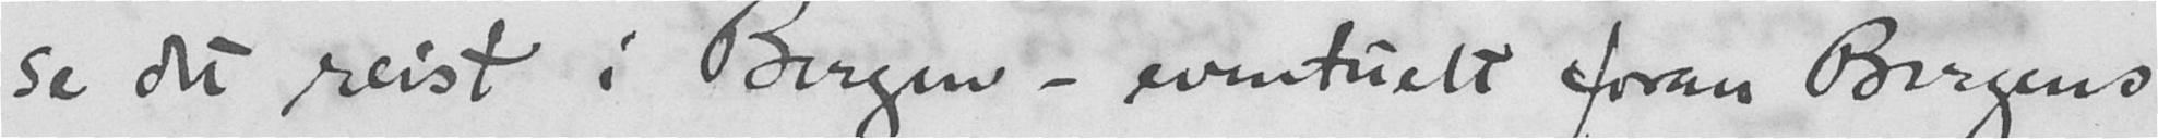se det reist i Bergen - eventuelt foran Bergens
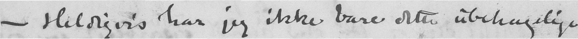- Heldigvis har jeg ikke bare dette ubehagelige
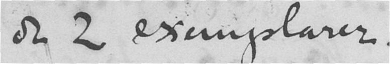de 2 exemplarerer.
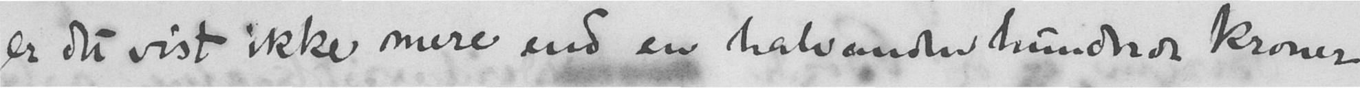er det vist ikke mere end en halvandenhundrede kroner
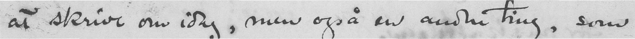at skrive om idag, men også en anden ting, som
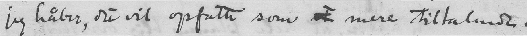jeg håber, du vil opfatte som et mere tiltalende.
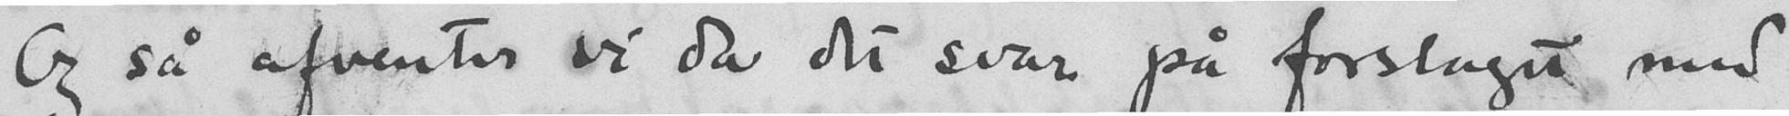Og så afventer vi da det svar på forslaget med
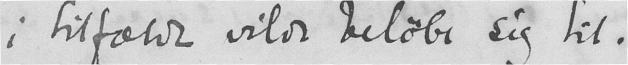i tilfælde vilde beløbe sig til.
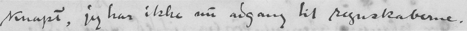knapt, jeg har ikke nu adgang til regnskaberne.
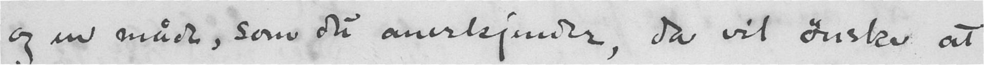og en måde, som du anerkjender, da vil ønske at
